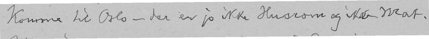Komme til Oslo - der er jo ikke Husrom og ikke Mat.
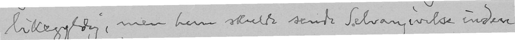likegyldig, men hun skulde sende Selvangivelse inden
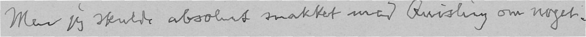Men jeg skulde absolut snakket med Quisling om noget.
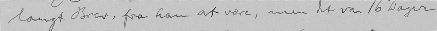langt Brev, fra ham at være, men det var 16 Dager
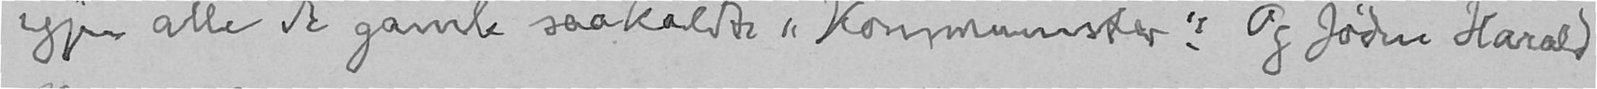igjen alle de gamle saakaldte "Kommunister". Og Jøden Harald
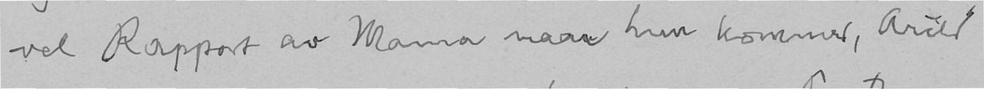vel Rapport av Mama naar hun kommer, Arild
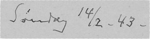Søndag 14/2. 43.
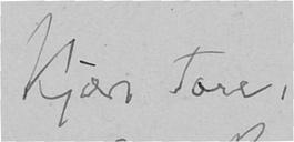Kjære Tore,
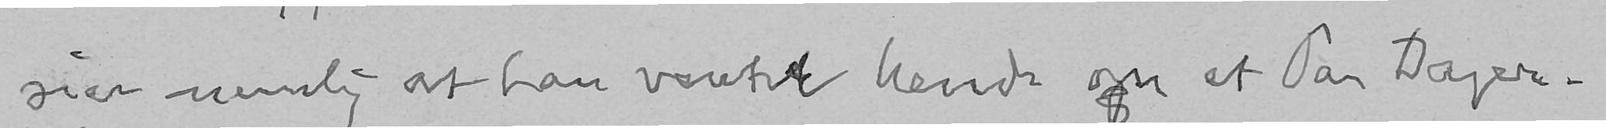sier nemlig at han venter hende om et Par Dager.
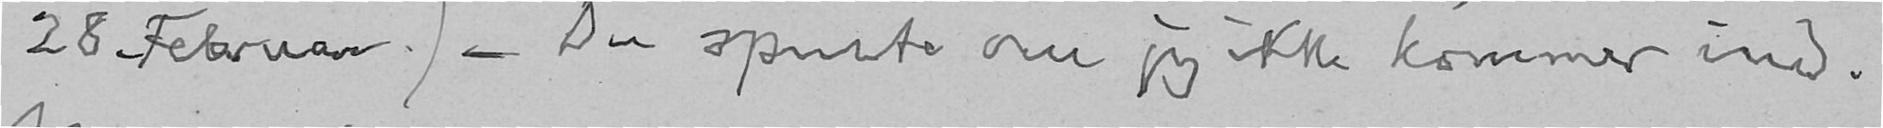28. Februar.) - Du spurte om jeg ikke kommer ind.
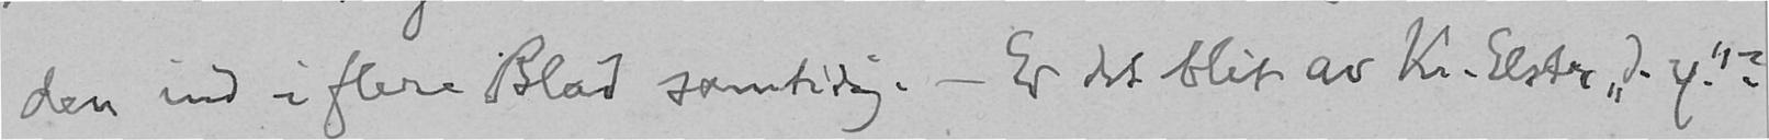den ind i flere Blad samtidig. - Er det blit av Kr. Elster "d. y."?
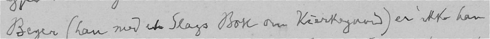Beyer (han med et Slags Bok om Kierkegaard) er ikke han
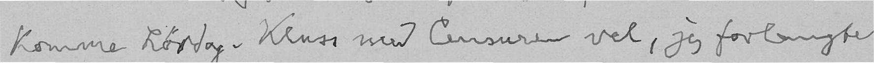komme Lørdag. Kluss med Censuren vel, jeg forlangte
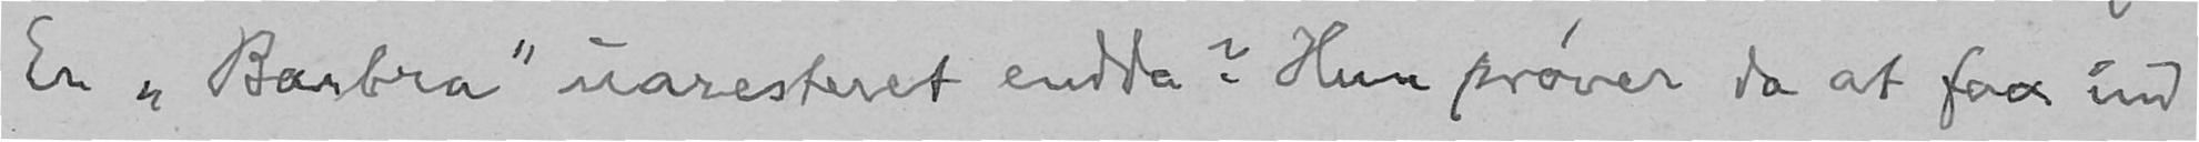Er "Barbra" uaresteret endda? Hun prøver da at faa ind
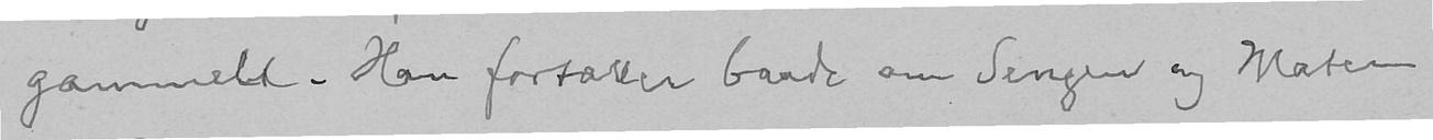gammelt. Han fortæller baade om Sengen og Maten
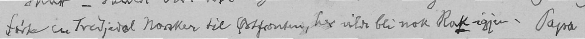førte en Tredjedel Norsker til Østfronten, her vilde bli nok Rak igjen. Papa
